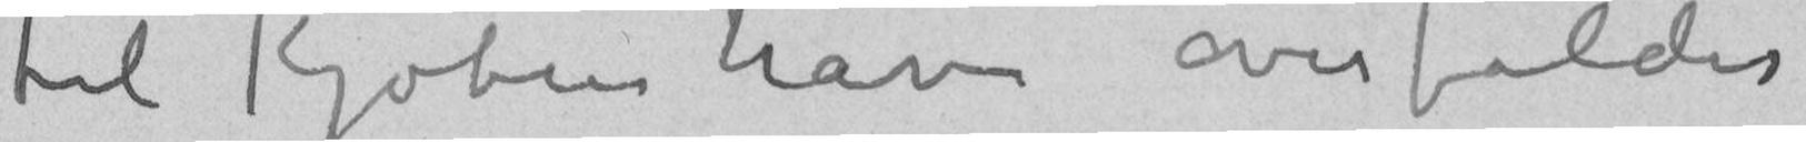til Kjøbenhavn overfaldes
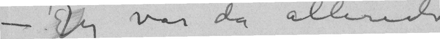– Jeg var da allerede
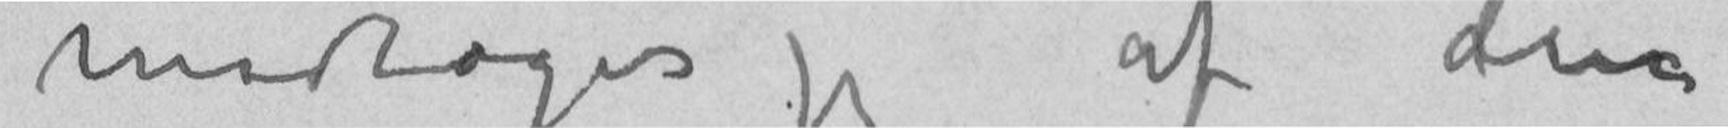modtoges jeg af den
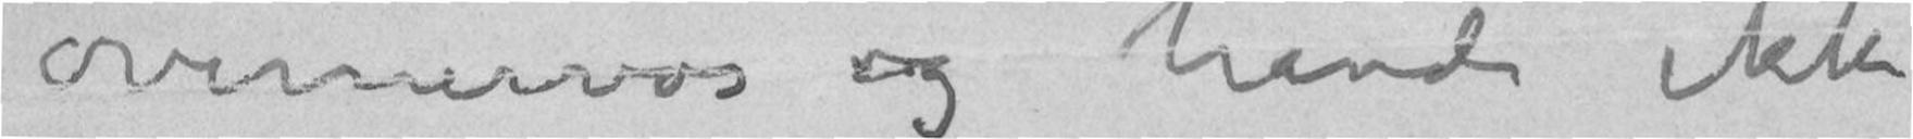overnervøs og havde ikke
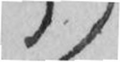5)
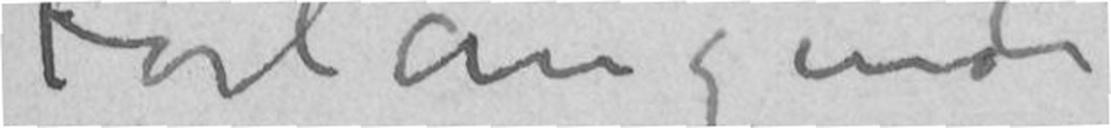Forlangende
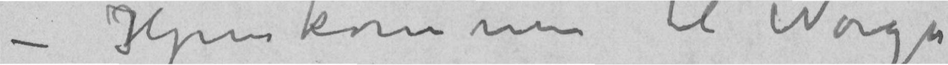– Hjemkommen til Norge
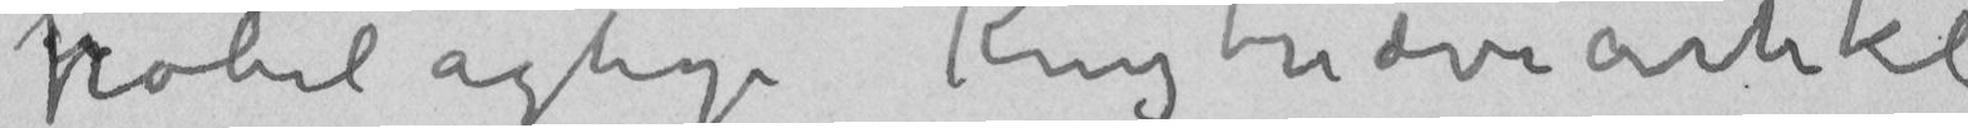pøbelagtige Knytnæveartikel
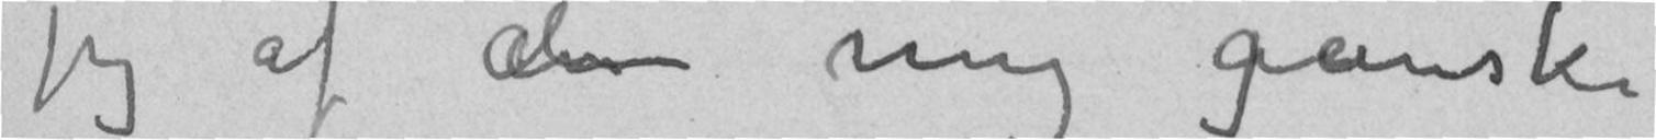jeg af den mig ganske
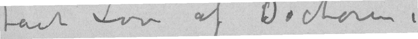fået Lov af Doctoren i
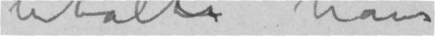betalt hans
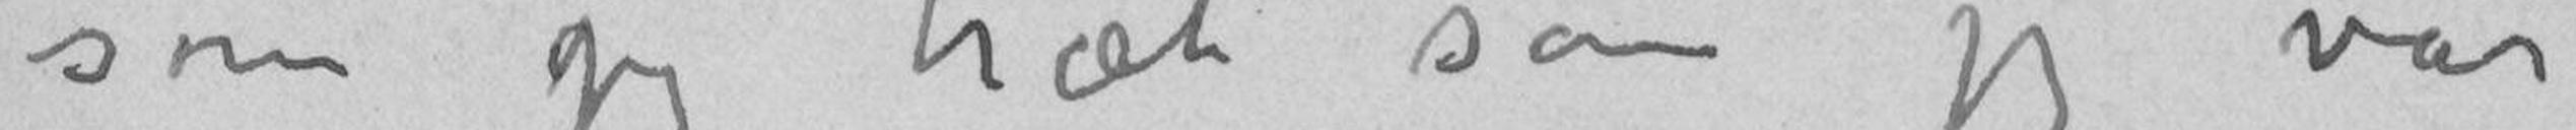som jeg træt som jeg var
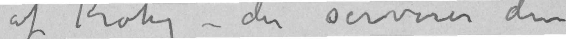af Krohg – der serverer den
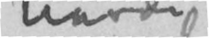havde
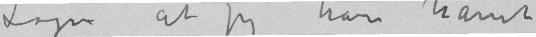Løgn at jeg have hånet
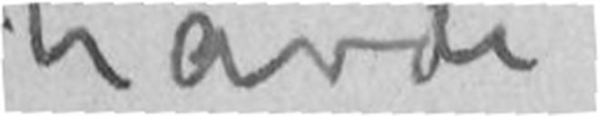havde
